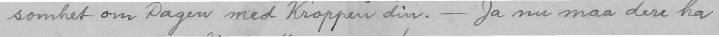somhet om Dagen med Kroppen din. - Ja nu maa dere ha
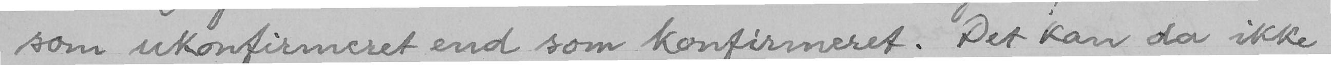som ukonfirmeret end som konfirmeret. Det kan da ikke
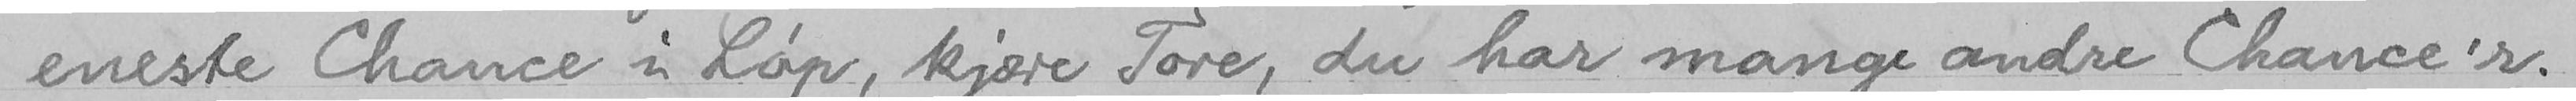eneste Chance i Løp, kjære Tore, du har mange andre Chance'r.
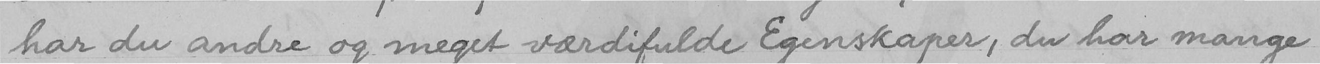har du andre og meget værdifulde Egenskaper, du har mange
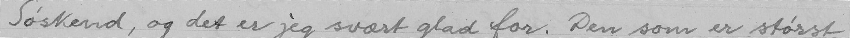Søskend, og det er jeg svært glad for. Den som er størst
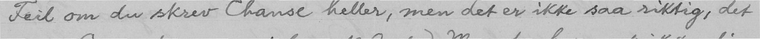Feil om du skrev Chanse heller, men det er ikke saa riktig, det
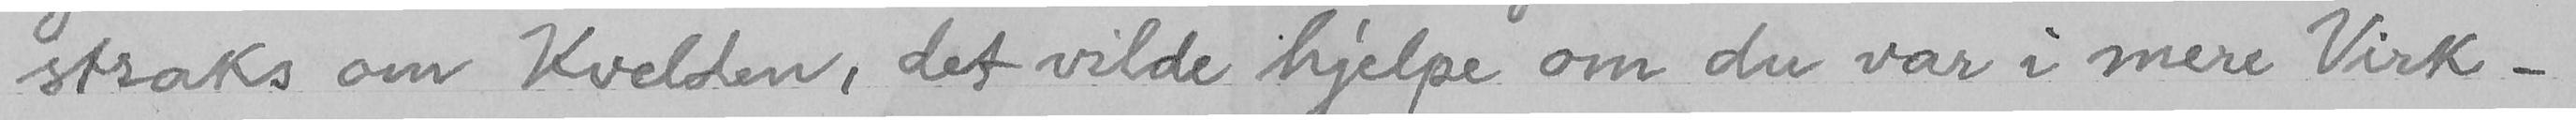straks om Kvelden, det vilde hjelpe om du var i mere Virk-
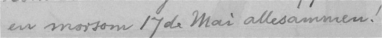en morsom 17de Mai allesammen!
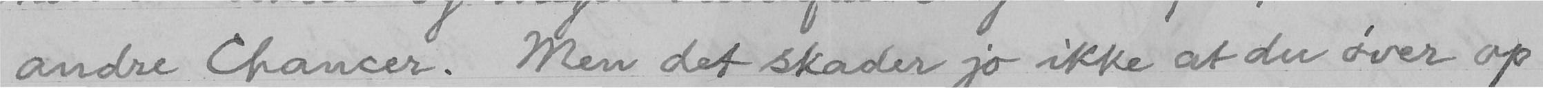andre Chancer. Men det skader jo ikke at du øver op
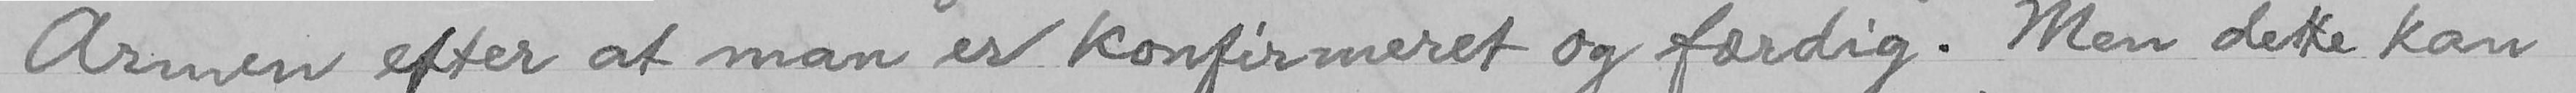Armen efter at man er konfirmeret og færdig. Men dette kan
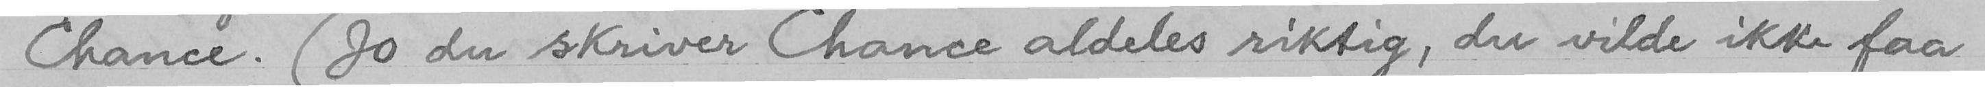Chance. (Jo du skriver Chance aldeles riktig, du vilde ikke faa
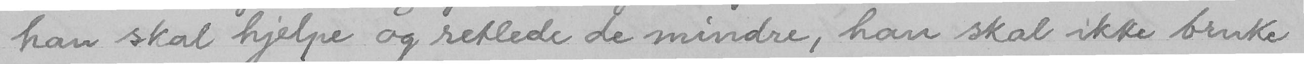han skal hjelpe og retlede de mindre, han skal ikke bruke
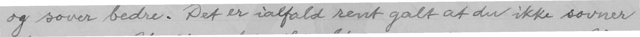og sover bedre. Det er ialfald rent galt at du ikke sovner
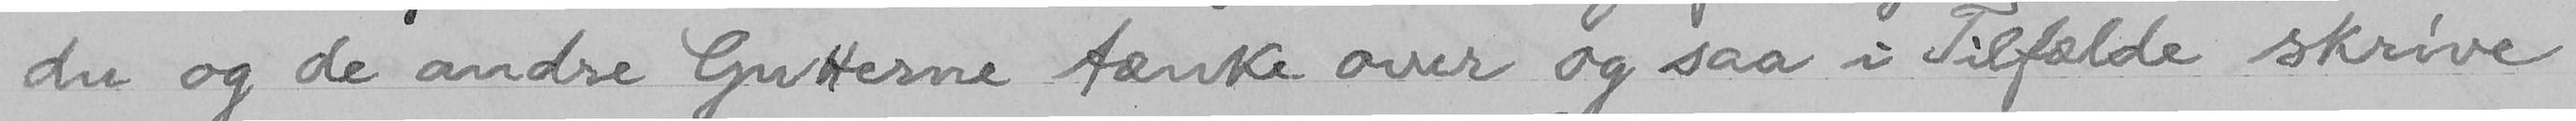du og de andre Gutterne tænke over og saa i Tilfælde skrive
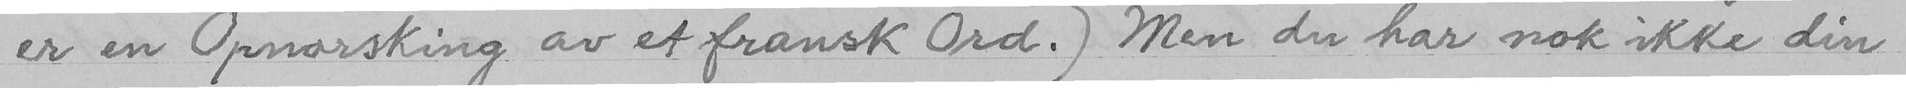er en Opnorsking av et fransk Ord.) Men du har nok ikke din
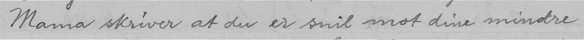Mama skriver at du er snil mot dine mindre
